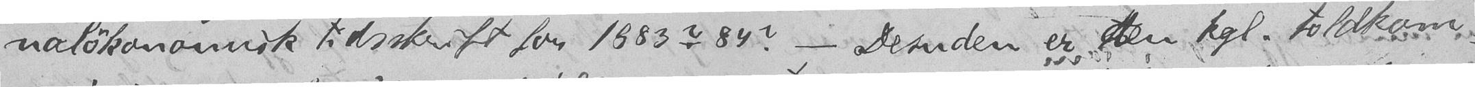naløkonomisk tidsskrift for 1883? 84? - Desuden er den kgl. toldkom-
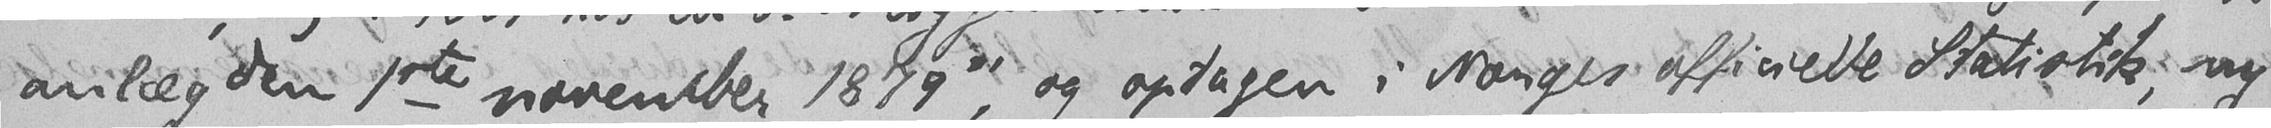anlæg den 1ste november 1879", og optagen i Norges officielle Statistik, ny
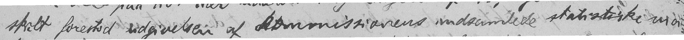skilt forestod udgivelsen af kommissionens indsamlede statistiske mo-
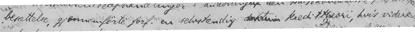besættelse, gjennemførte forf. en selvstændig  kredittheori, hvis videre
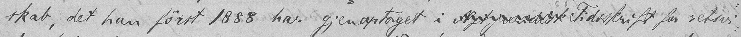skab, det han først 1888 har gjenoptaget i  Tidsskrift for retsvi-
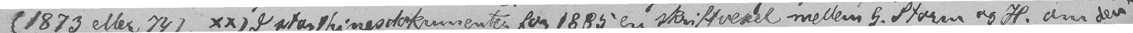(1873 eller 74). xx) I stothingsdokumenter for 1885 en skriftvexel mellem G. Storm og H. om den
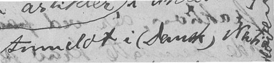Anmeldt i (Dansk) Natio-
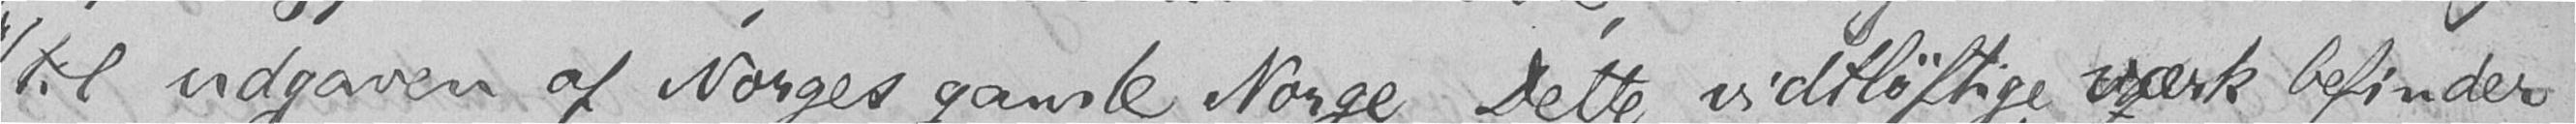til udgaven af Norges gamle Norge. Dette vidtløftige værk befinder
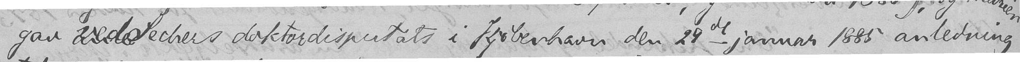gav ved Sechers doktordisputats i Kjøbenhavn den 29de januar 1885 anledning
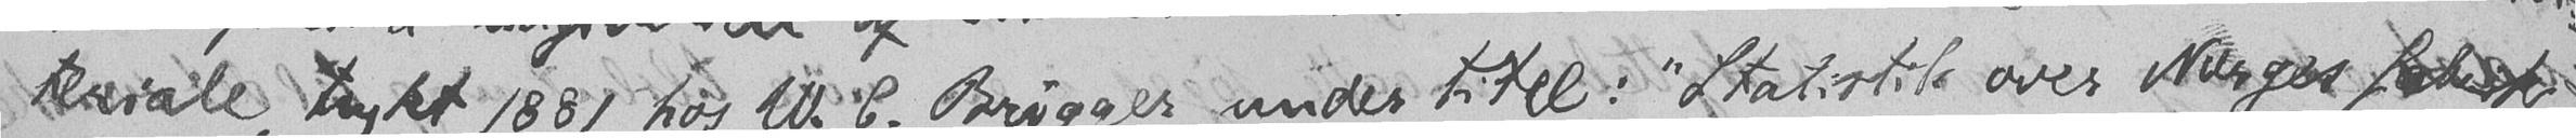teriale, trykt 1881 hos W. C. Brøgger under titel: "Statistik over Norges fabrik-
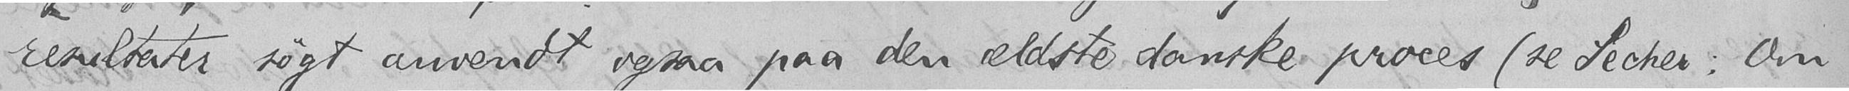resultater søgt anvendt ogsaa paa den ældste danske proces (se Secher: Om
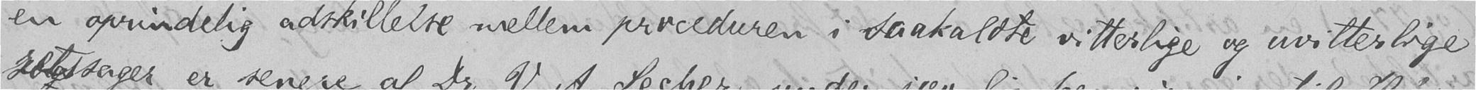en oprindelig adskillelse mellem proceduren i saakaldte vitterlige og uvitterlige
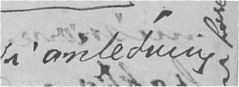i anledning
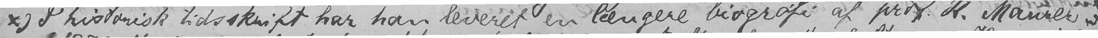x) I historisk tidsskrift har han leveret en længere biografi af prof. K. Maurer
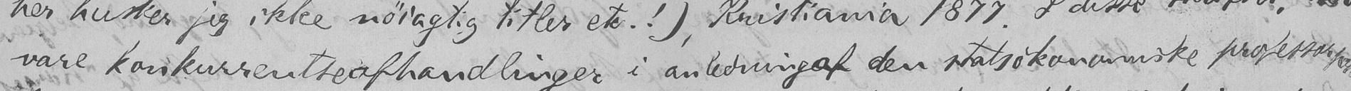vare konkurrentseafhandlinger i anledning af den statsøkonomiske professorposts
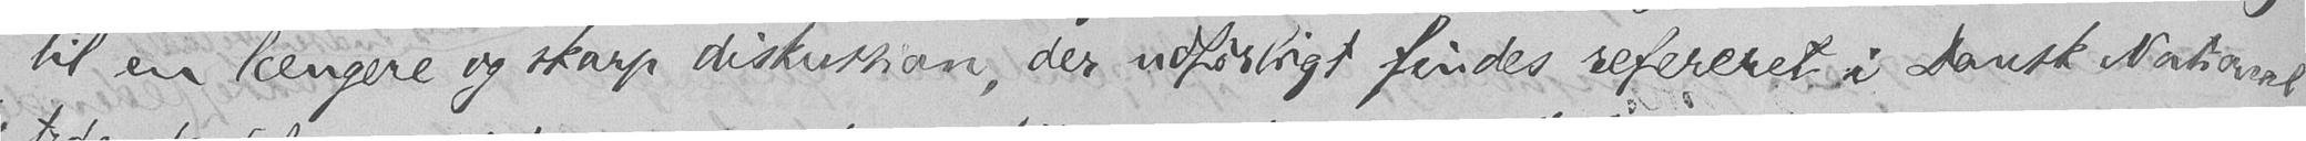til en længere og skarp diskussion, der udførligt findes refereret i Dansk National-
